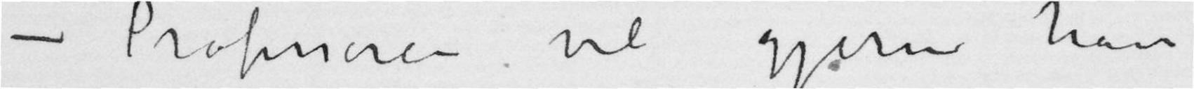– Professoren vil gjerne have
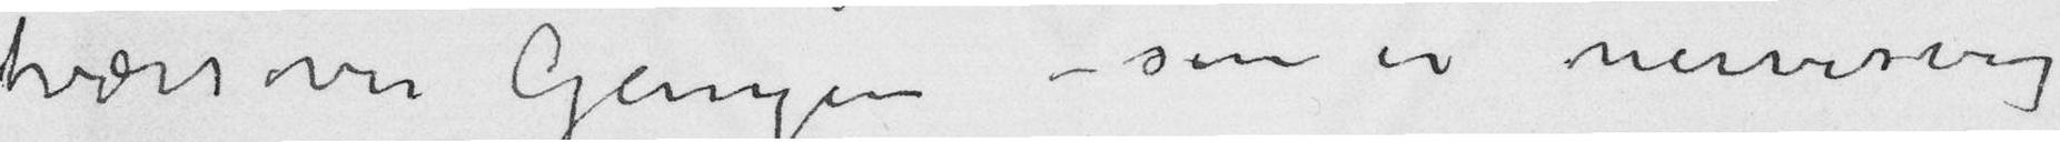tværs over Gangen – som er nervesvag
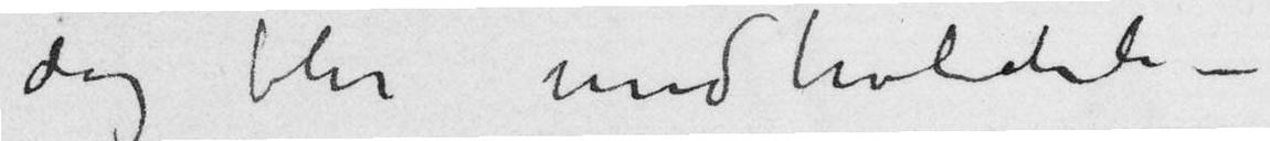dog blir uudholdeli –
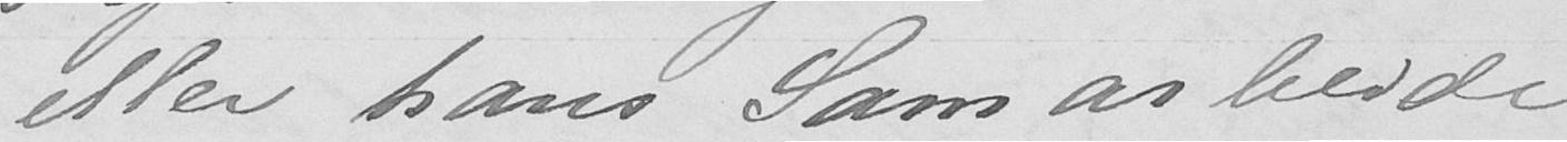eller hans Samarbeide
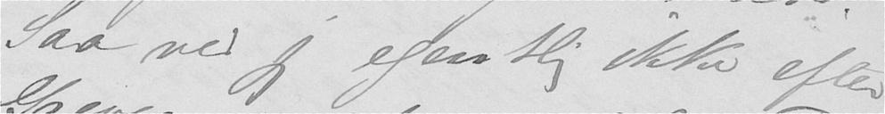Saa ved jeg egentlig ikke efter
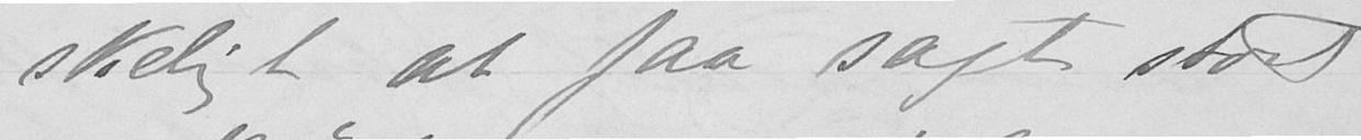skeligt at faa sagt stort
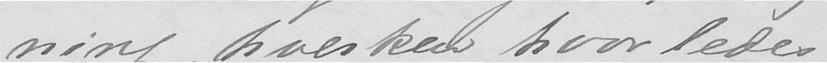ning, hverken hvorledes
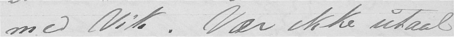med Vik. Vær ikke utaal-
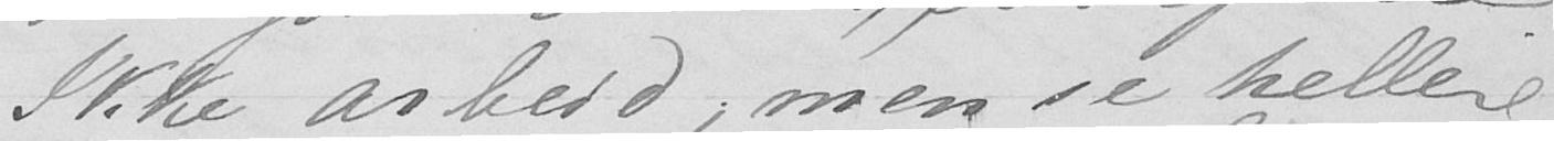Ikke arbeid, men se heller
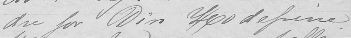dre for Din Hodepine.
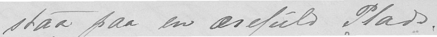staa paa en ærefuld Plads.
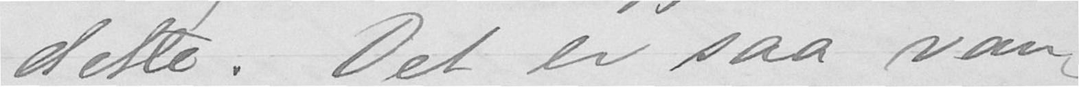dette. Det er saa van-
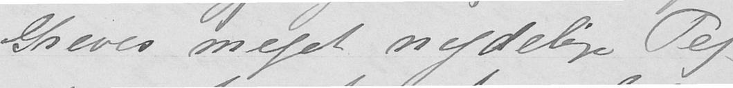Greves meget nydelige Teg-
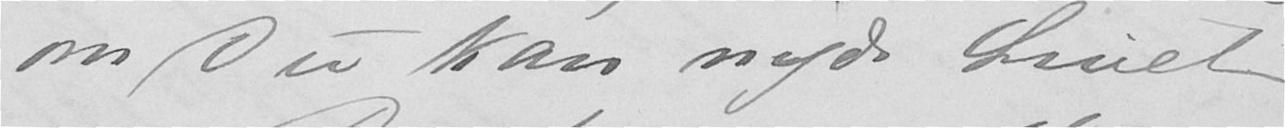om Du kan nyde Livet -
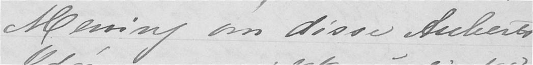Mening om disse Auberts
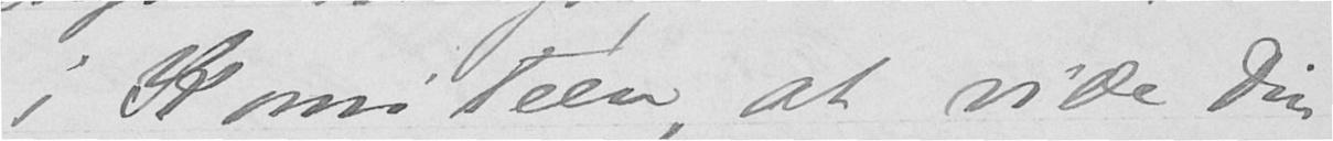i Komitéen, at vide din
